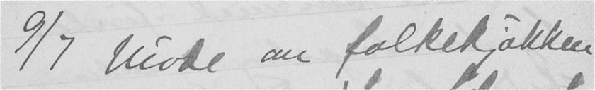9/7 Møde om folkekjøkken
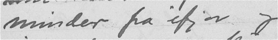minder fra ifjor og
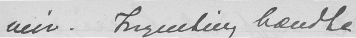veir. Ingenting hændte
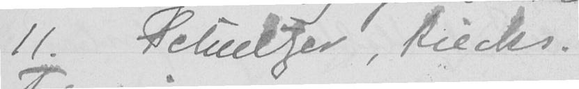11. Schultzer, Riecks.
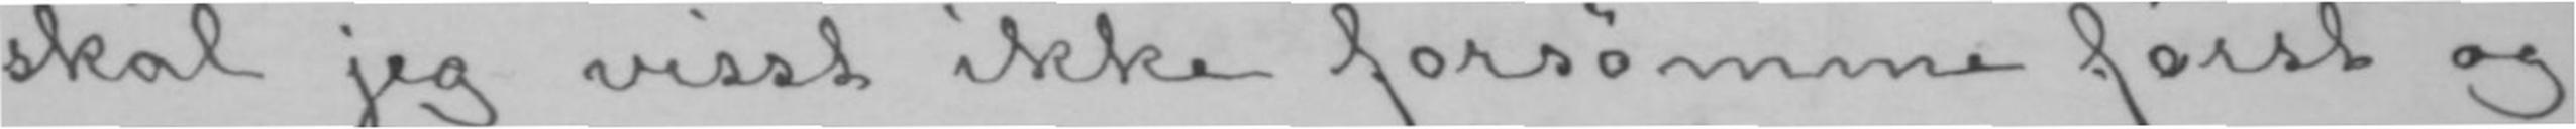skal jeg visst ikke forsømme først og
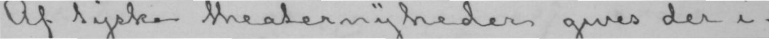Af tyske theaternyheder gives der i-
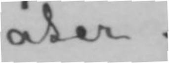ater.
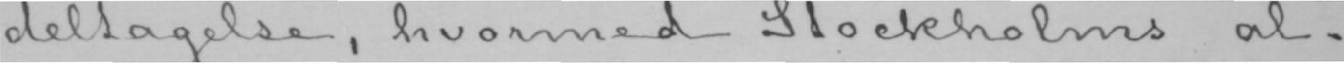deltagelse, hvormed Stockholms al-
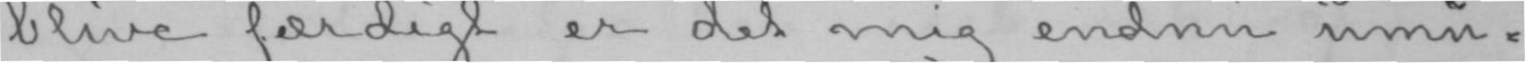blive færdigt er det mig endnu umu-
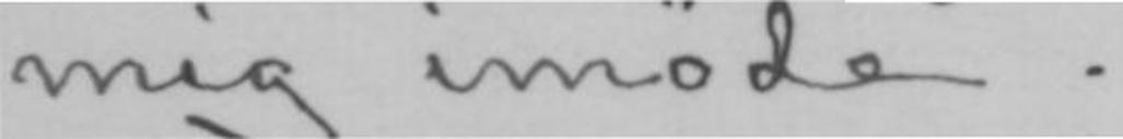mig imøde.
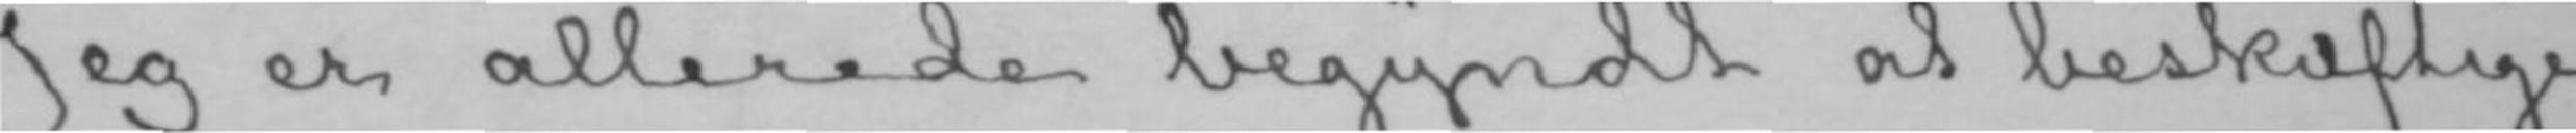Jeg er allerede begyndt at beskæftige
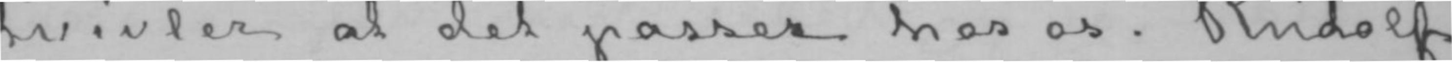tvivler at det passer hos os. Rudolf
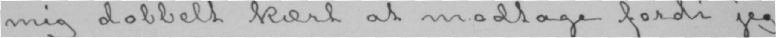mig dobbelt kært at modtage fordi jeg
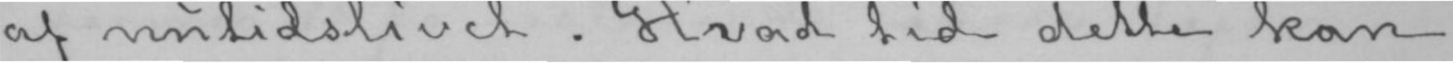af nutidslivet. Hvad tid dette kan
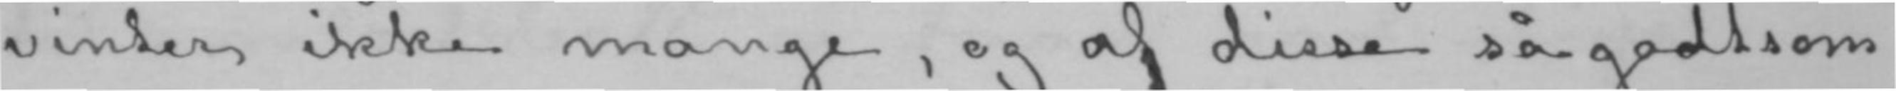vinter ikke mange, og af disse sågodtsom
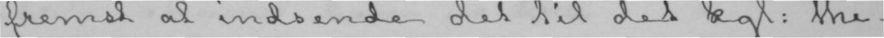fremst at indsende det til det kgl: the-
